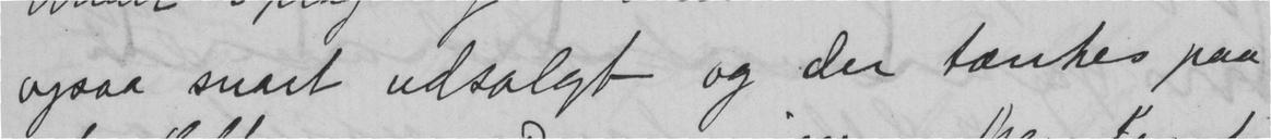ogsaa snart udsolgt og der tænkes paa
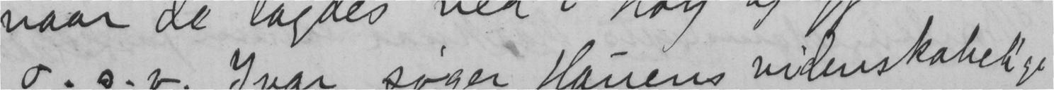o. s. v. Ivar søger Hauens videnskabelige
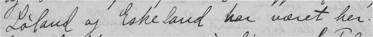Løland og Eskeland har været her.
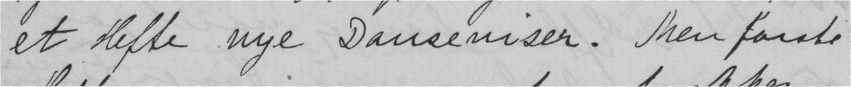et Hefte nye Danseviser. Men første
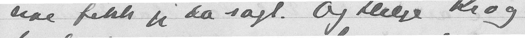noe fikk jeg da sagt. Og Helge Krog
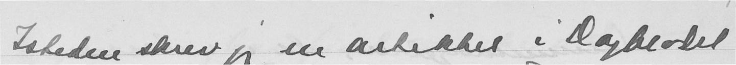Isteden skrev jeg en artikkel i Dagbladet
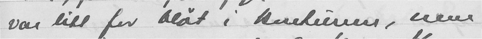var litt for bløt i konturene, men
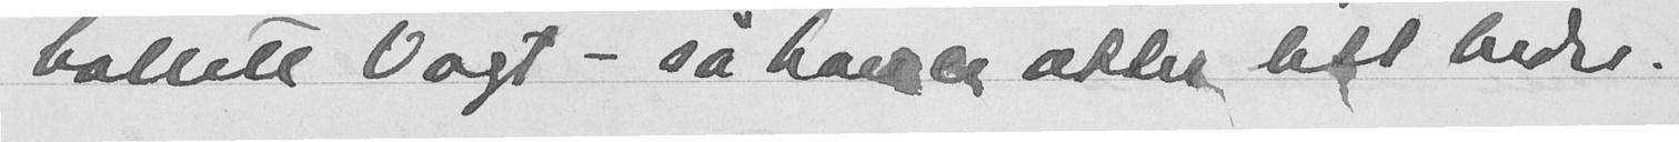Collett Vogt - så han er atter litt bedre.
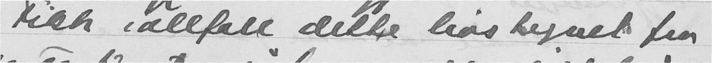Fikk iallfall dette livstegnet fra
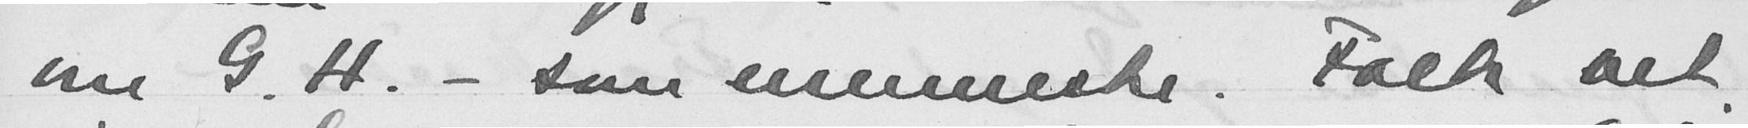om G. H. - som menneske. Folk vet
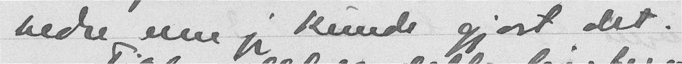bedre enn jeg kunde gjort det.
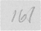161
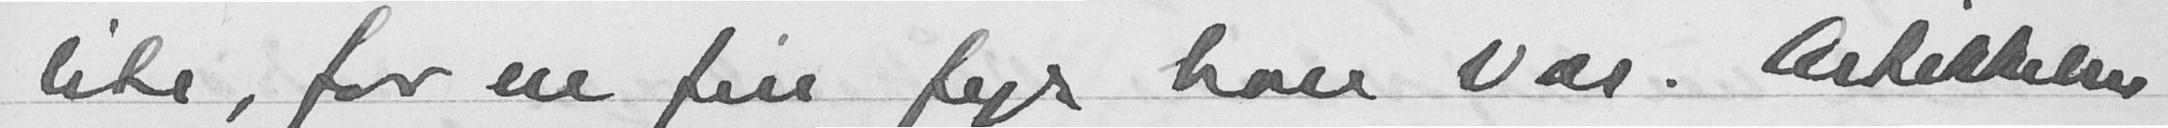lite, for en fin fyr han var. Artikkelen
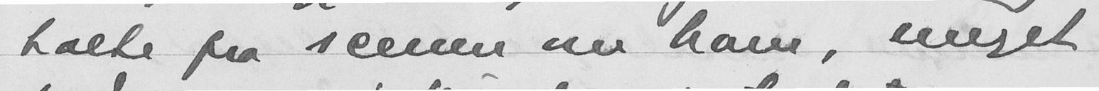talte fra scenen om ham, meget
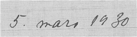5. mars 1930
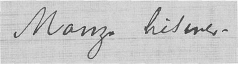Mange hilsener
